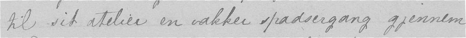til sit atelier en vakker spadsergang gjennem
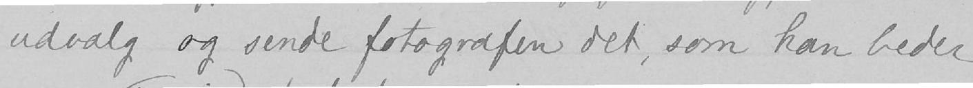udvalg og sende fotografen det, som han beder
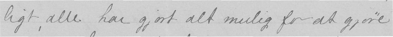ligt, alle har gjort alt mulig for at gjøre
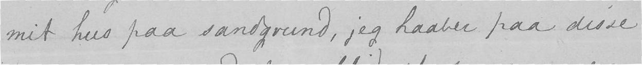mit hus paa sandgrund, jeg haaber paa disse
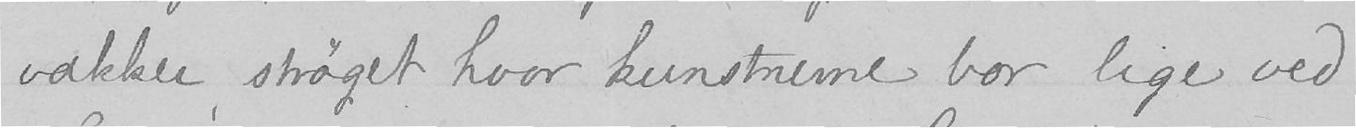vakker, strøget hvor kunstnerne bor lige ved
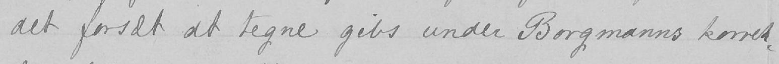det forsæt at tegne gibs under Borgmanns korrek-
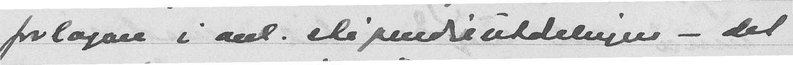forlagen i anl. stipindiutdelingen - det
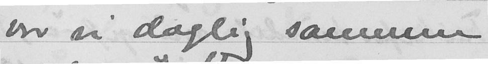var vi daglig sammen
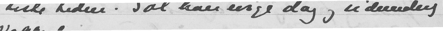siste tiden. Sol hver evige dag og vidunderlig
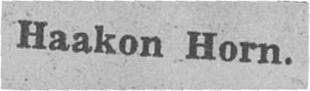Haakon Horn.
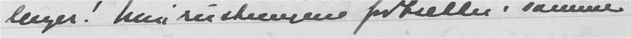lenger. Men rustningene fortsetter i samme
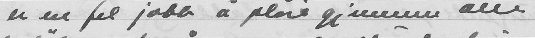er en fel jobb å pløie gjennom alle
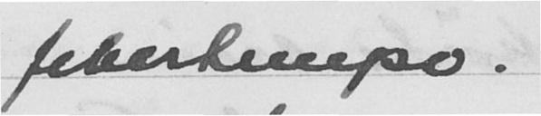febertempo.
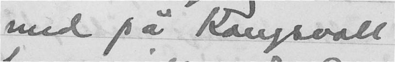med på Kongsvoll
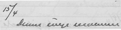15/4 Denne unge mann
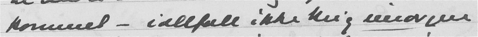kommet - iallfall ikke krig imorgen
